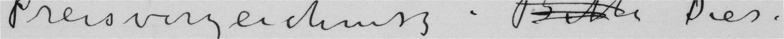Preisverzeichnisz. Bitte Diese
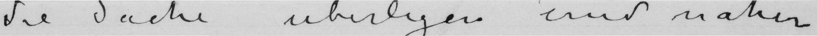die Sache uberlegen und naher
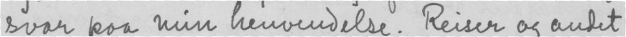svar paa min henvendelse. Reiser og andet
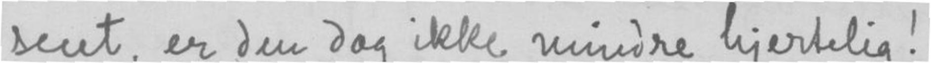sent, er den dog ikke mindre hjertelig!
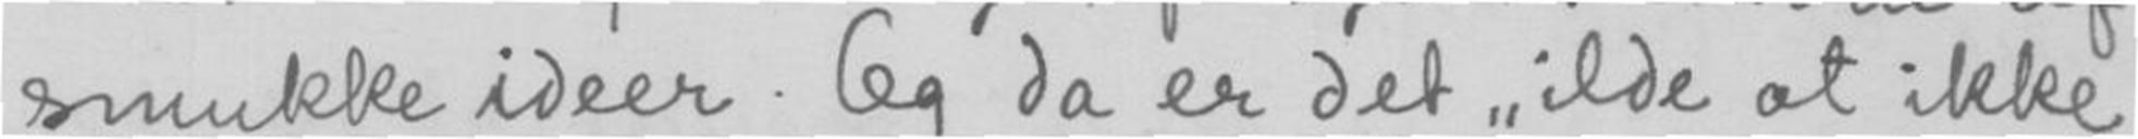smukke ideer. Og da er det ilde at ikke
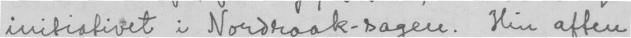initiativet i Nordraach-sagen. Hin aften
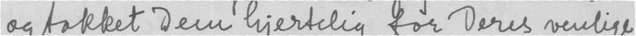og takket Dem hjertelig for Deres venlige
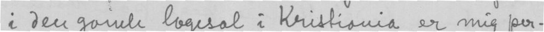i den gamle logesal i Kristiania er mig per-
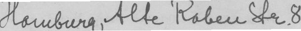Hamburg, Alte Raben Str.8
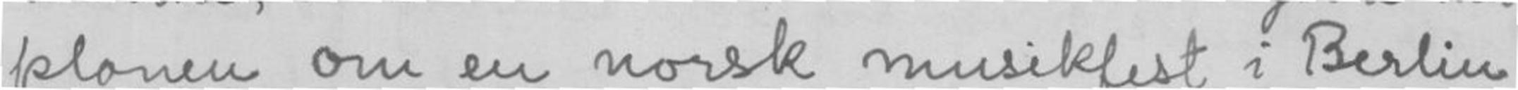planen om en norsk musikfest i Berlin
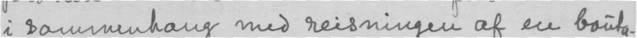i sammenhæng med reisningen af en bauta-
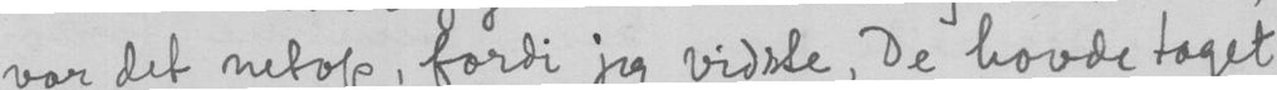var det netop, fordi jeg vidste, De havde taget
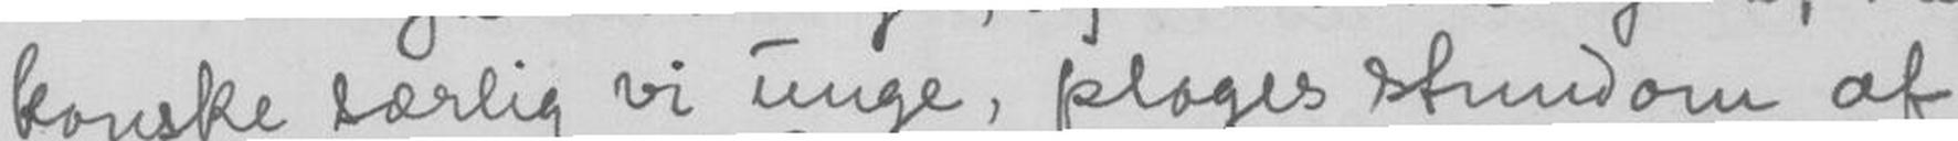kanske særlig vi unge, plages stundom af
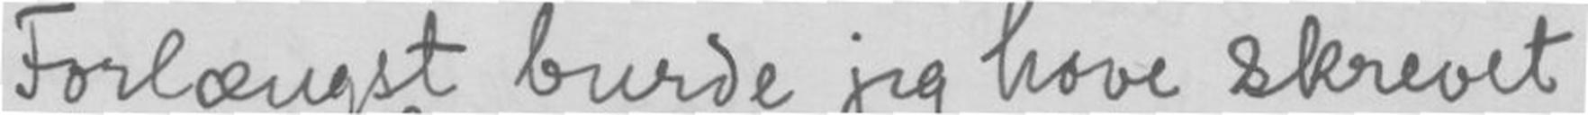Forlængst burde jeg have skrevet
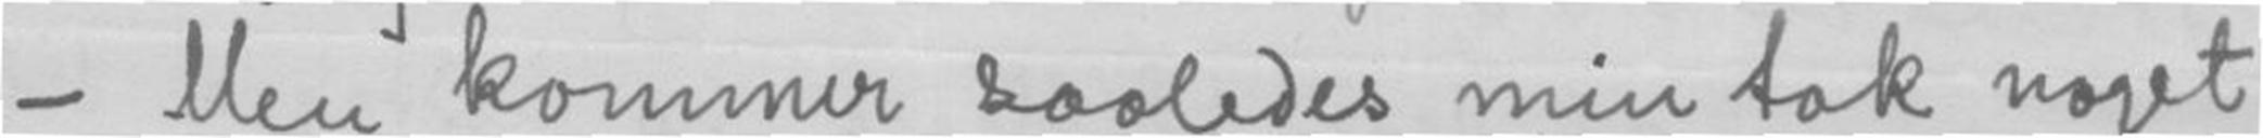- Men kommer saaledes min tak noget
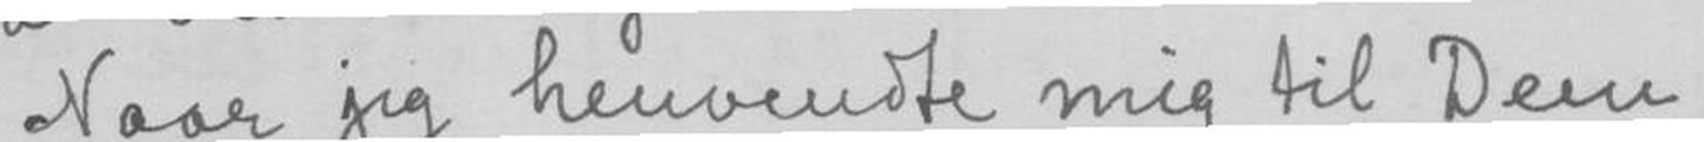Naar jeg henvendte mig til Dem,
